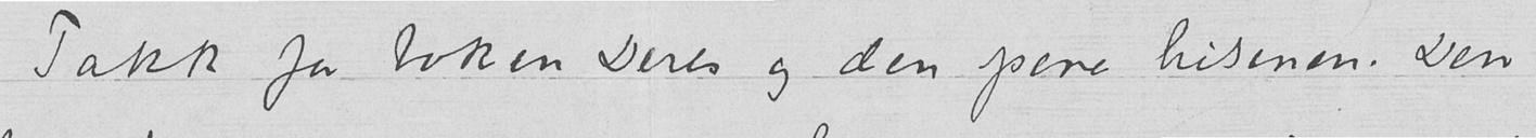Takk for boken Deres og den pene hilsenen. Den
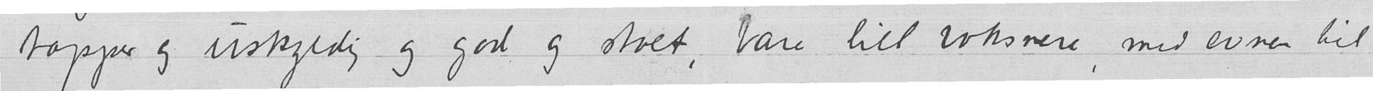tapper og uskyldig og god og stolt, bare litt voksnere, med evnen til
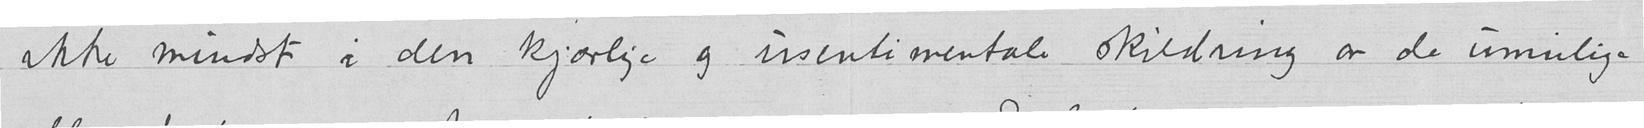ikke mindst i den kjærlige og usentimentale skildring av de umulige
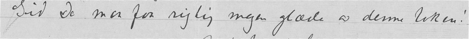Gid De maa faa rigtig megen glæde av denne boken!
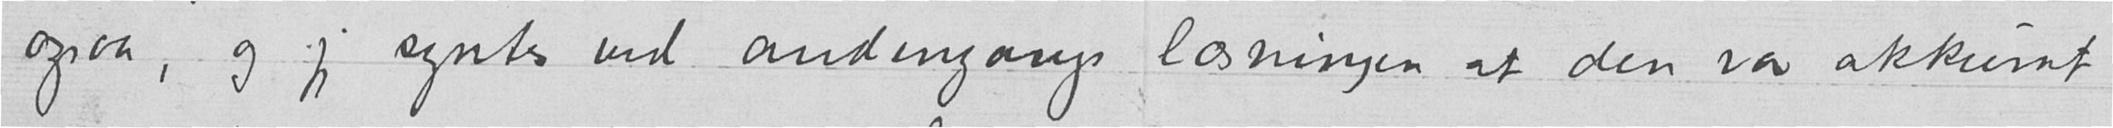ogsaa, og jeg syntes ved andengangs læsningen at den var akkurat
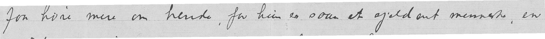faa høre mere om hende, for hun er saan et sjeldent menneske, en
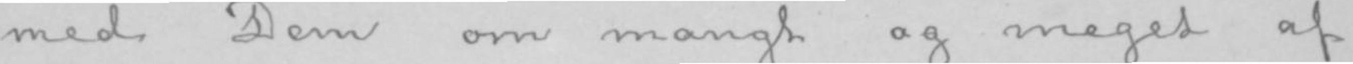med Dem om mangt og meget af
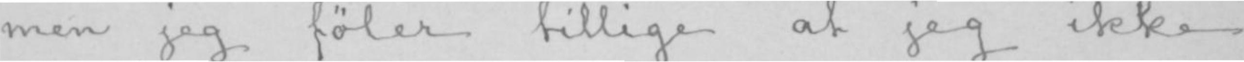men jeg föler tillige at jeg ikke
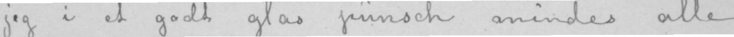jeg i et godt glas punsch mindes alle
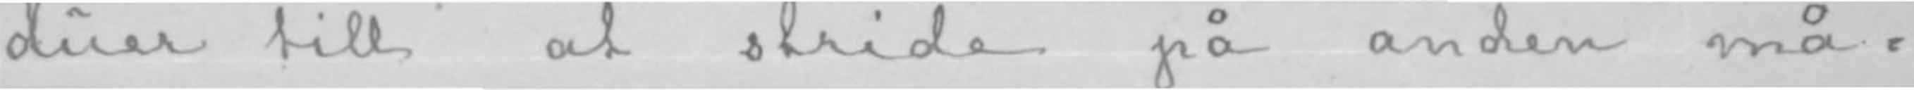duer till at stride på anden må-
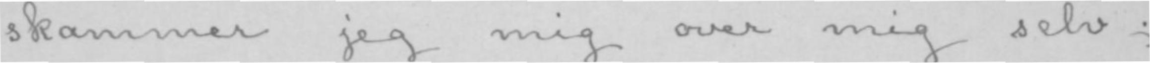skammer jeg mig over mig selv;
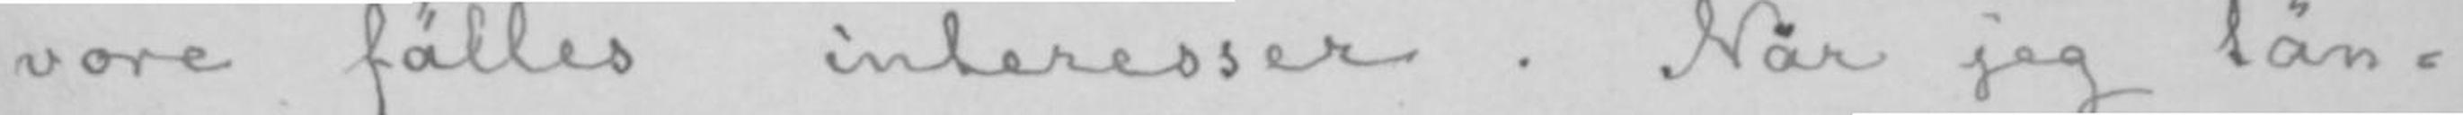vore fälles interesser. Når jeg tän-
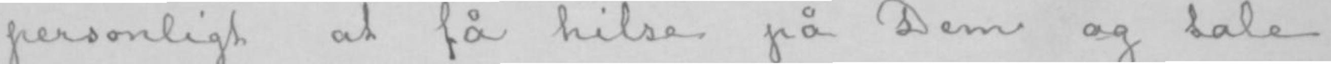personligt at få hilse på Dem og tale
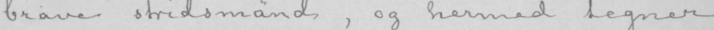brave stridsmänd, og hermed tegner
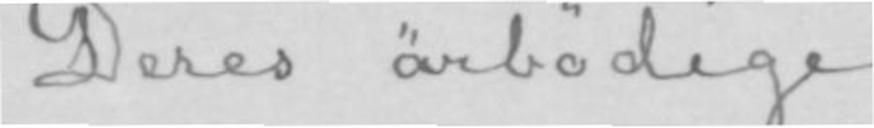Deres ärbödige
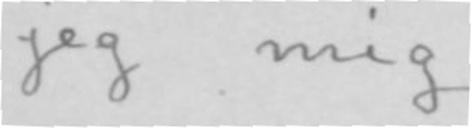jeg mig
